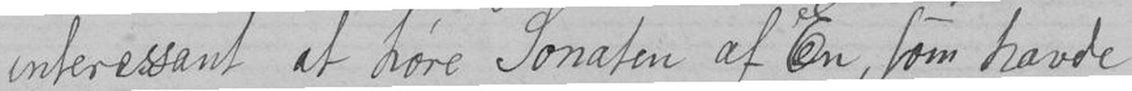interessant at høre Sonaten af En, som havde
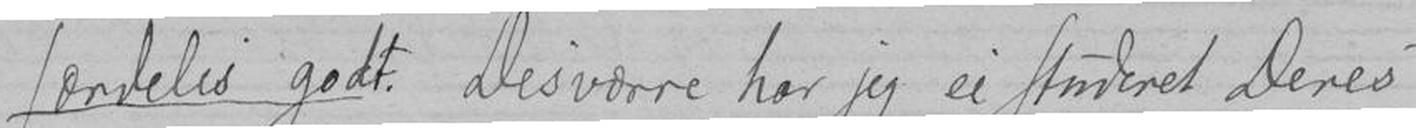særdeles godt. Desværre har jeg ei studeret Deres
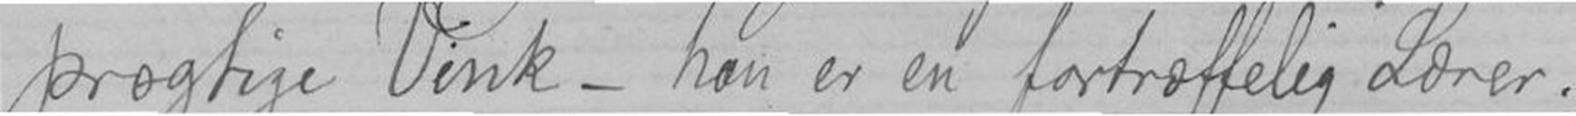prægtige Vink - han er en fortræffelig Lærer.
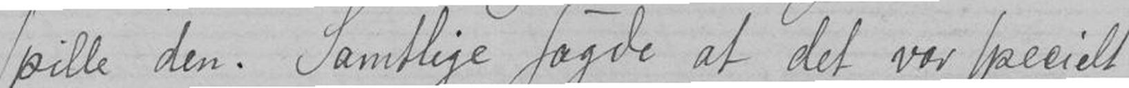spille den. Samtlige sagde at det var specielt
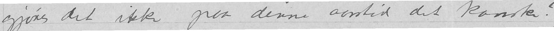gjøres det ikke paa denne aarstid det kanske?)
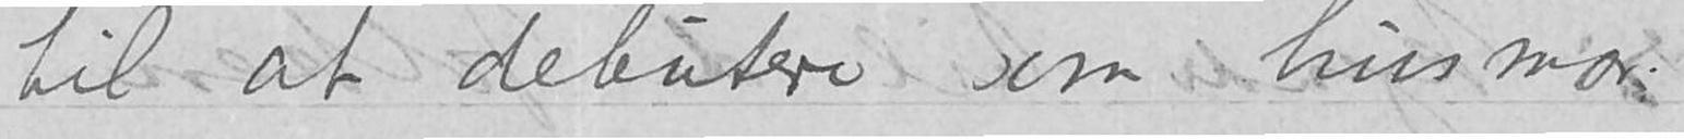til at debutere som husmor.
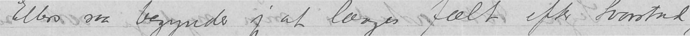Ellers saa begynder jeg at længes fælt efter Svarstad,
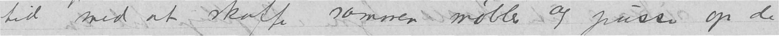tid med at skaffe sammen møbler og pusser op de
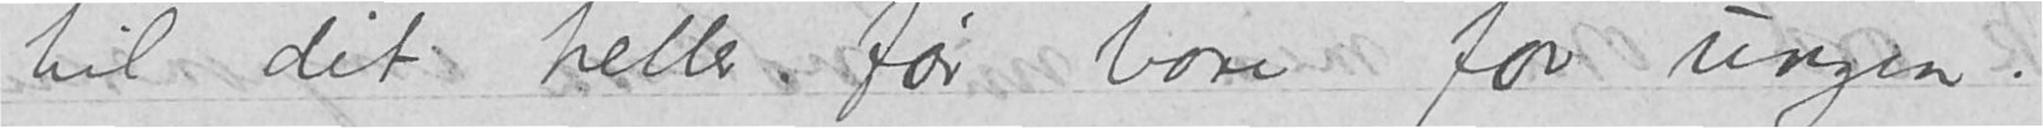til dit heller før bare for ungen.
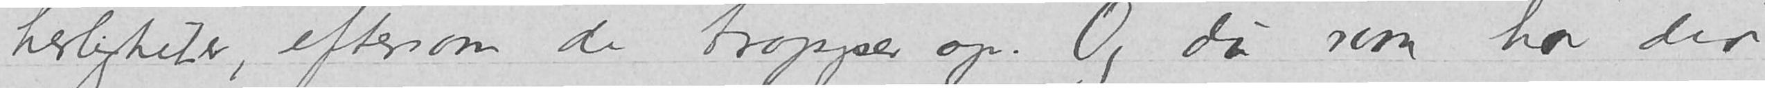herligheter, eftersom de tropper op. Og du som har din
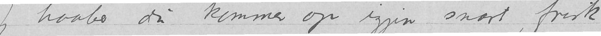Jeg haaber du kommer op igjen snart, frisk
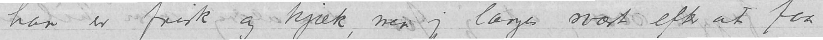han er frisk og kjæk, men jeg længes svært efter at faa
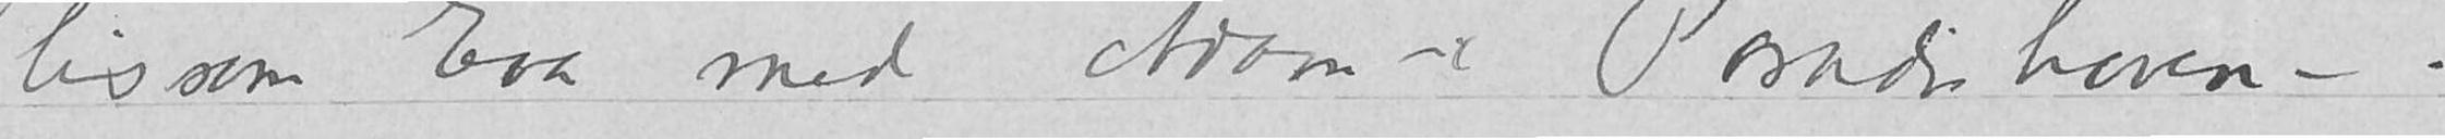lissom Eva med Adam i Paradishaven –.
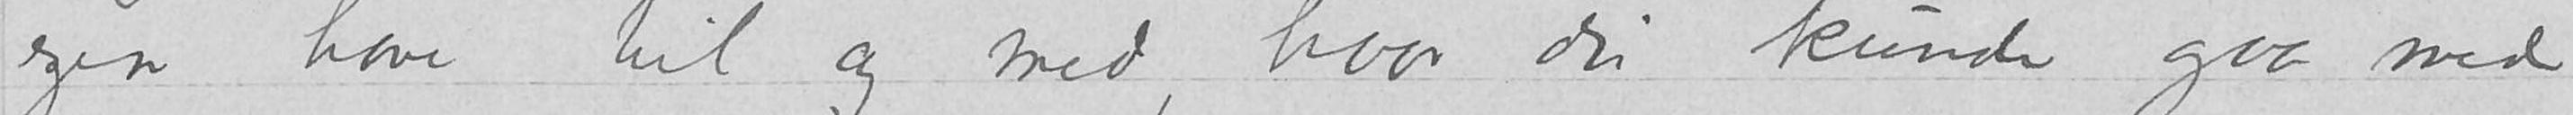egen have til og med, hvor du kunde gaa med
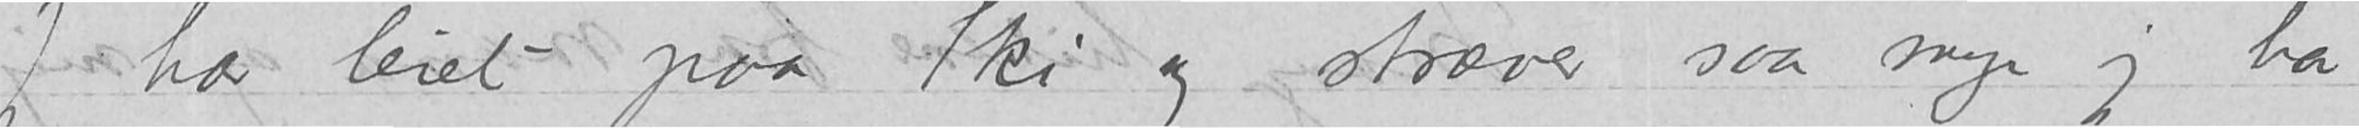Jeg har leiet – paa Ski og stræver saa mye jeg har
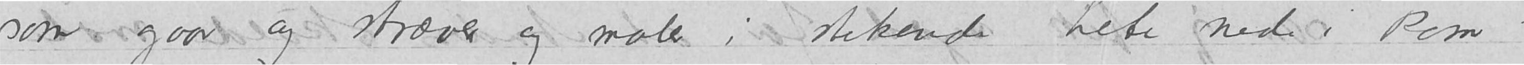som gaar og stræver og maler i stekende hete nede i Rom –
